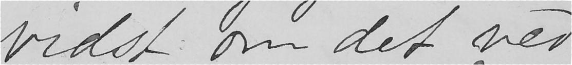vidst om det ved
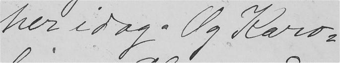her idag. Og Karo-
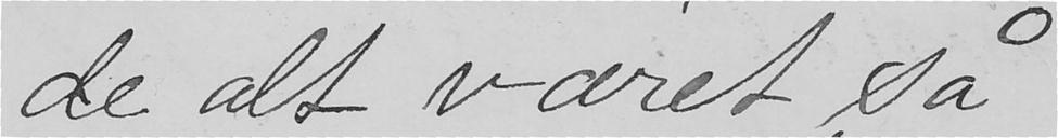de alt været så
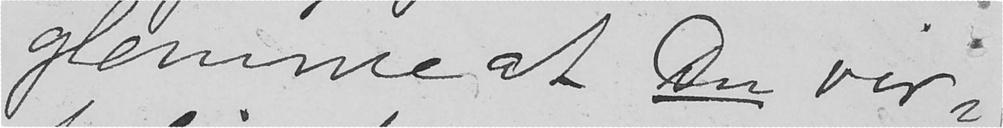gemme at Du vir-
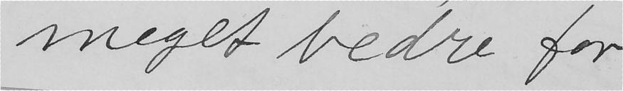meget bedre for
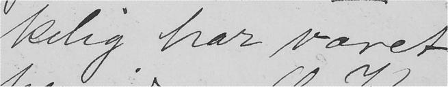kelig har været
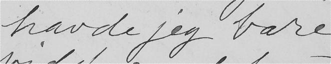havde jeg bare
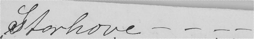Storhove - - - -
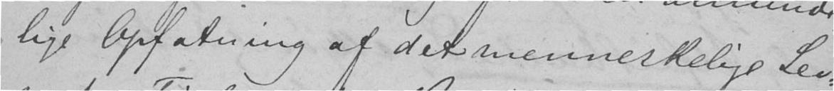lige Opfatning af det menneskelige Lev-
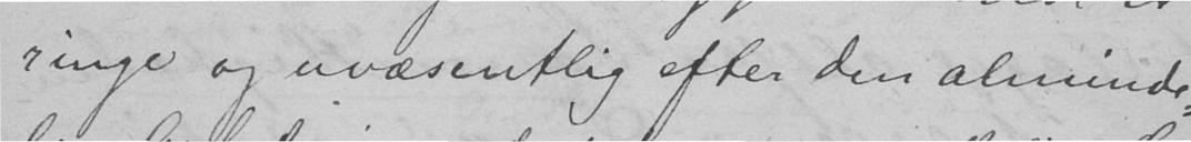ringe og uvæsentlig efter den alminde-
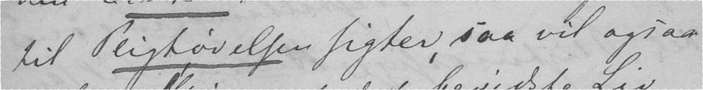til Pligtøvelsen sigter, saa vil ogsaa
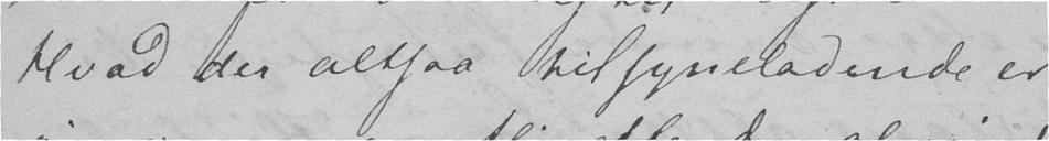Hvad der altsaa tilsyneladende er
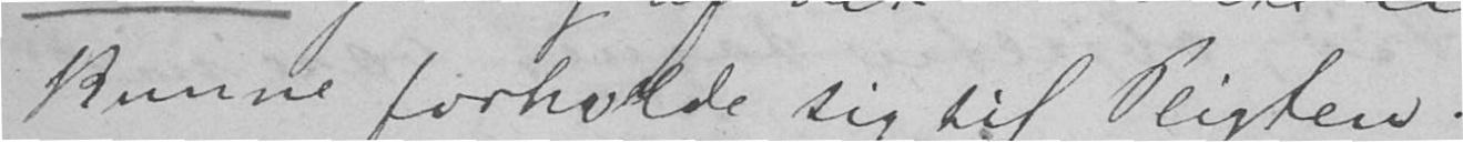kunne forholde sig til Pligten.
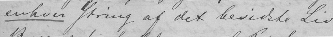enhver Ytring af det bevidste Liv
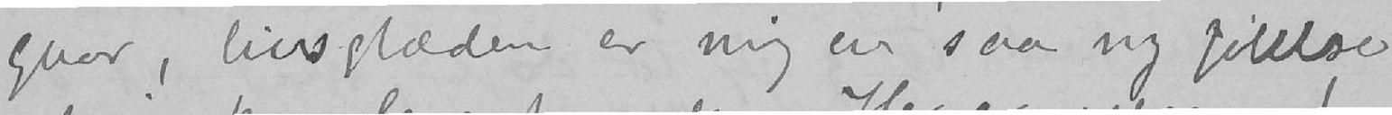gaar, livsglæden er mig en saa ny følelse
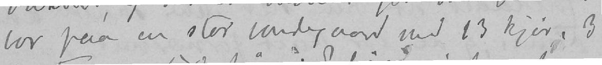bor på en stor bondegaard med 13 kjør, 3
